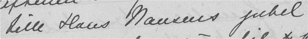Lille Hans Nansens jubel
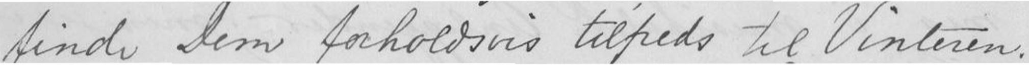finde Dem forholdsvis tilfreds til Vinteren;
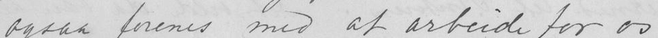ogsaa forenes med at arbeide for os
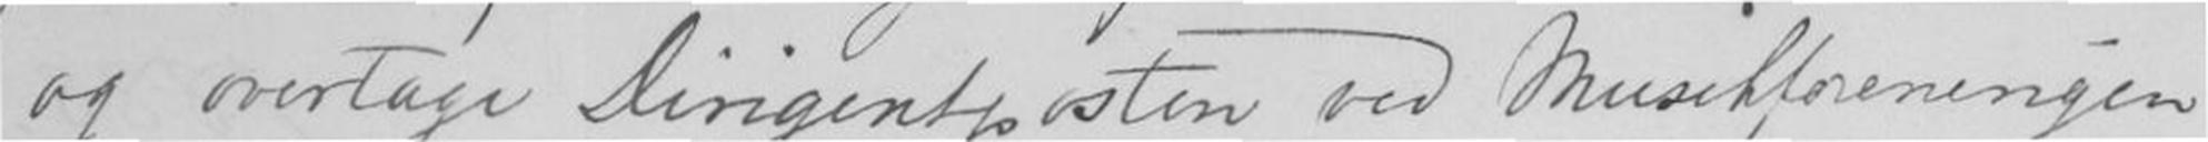og overtage Dirigentposten ved Musikforeningen
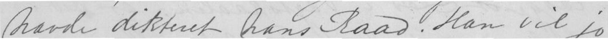havde dikteret hans Raad. Han vil jo
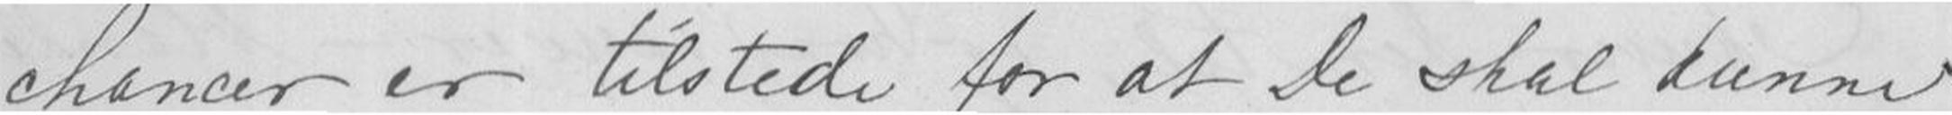chancer er tilstede for at De skal kunne
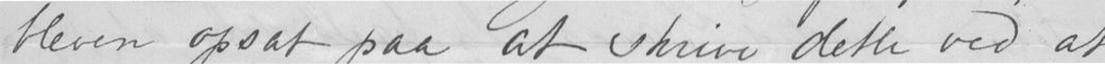bleven opsat paa at skrive dette ved at
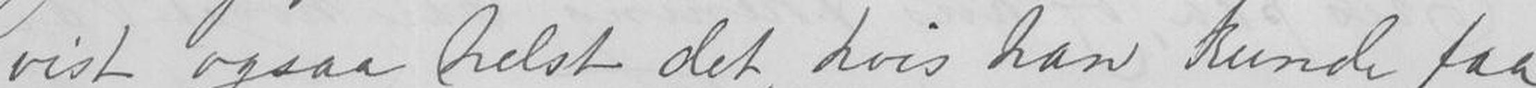vist ogsaa helst det, hvis han kunde faa
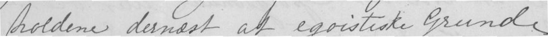holderne, dernest at egoistiske Grunde
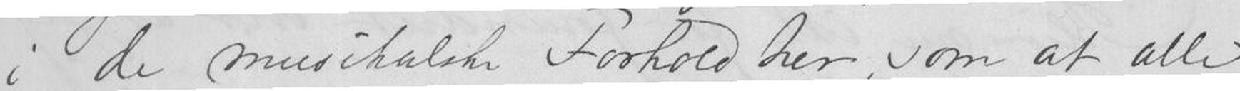i de musikalske Forhold her, som at alle
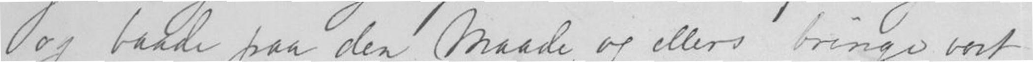og baade paa den Maade og ellers bringe vort
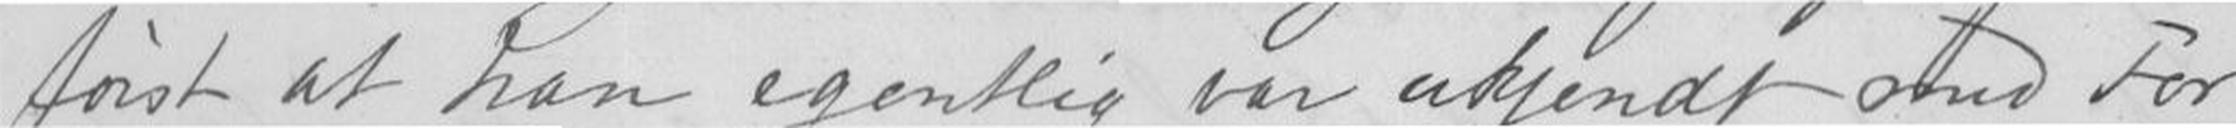først at han egentlig var ukjendt med For-
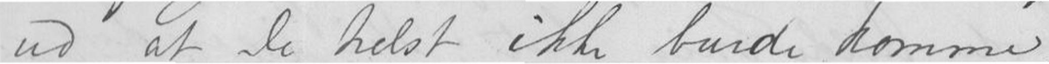ud at De helst ikke burde komme.
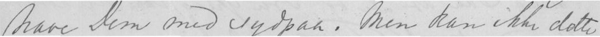have Dem med sydpaa. Men kan ikke dette
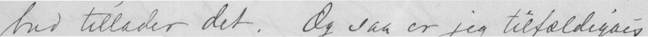bred tillader det. Og saa er jeg tilfældigvis
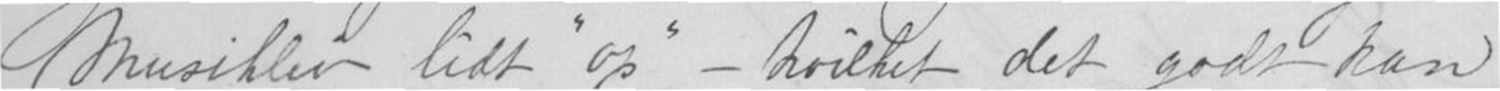Musikliv lidt "op" - hvilket det godt kan
